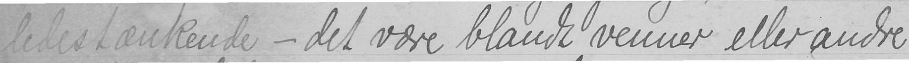ledestænkende - det være blandt venner eller andre -
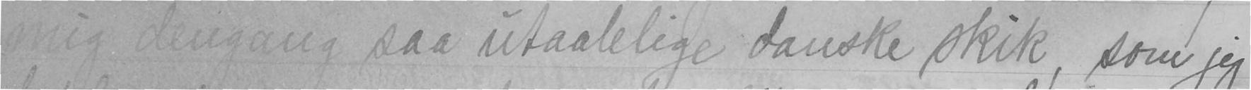mig dengang saa utaalelige danske skik, som jeg
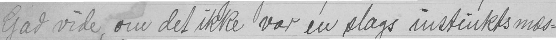Gud vide om det ikke var en slags instinktsmæs-
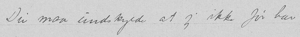Du maa undskylde at jeg ikke før har
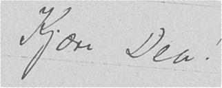Kjære Dea!
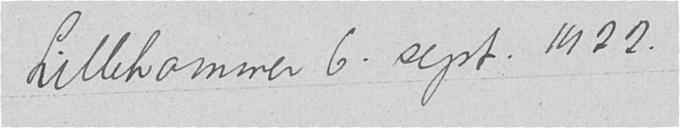Lillehammer 6. sept. 1922.
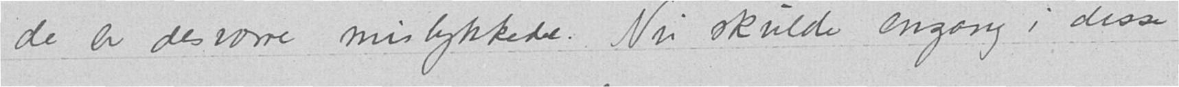de er desværre mislykkede. Nu skulde engang i disse
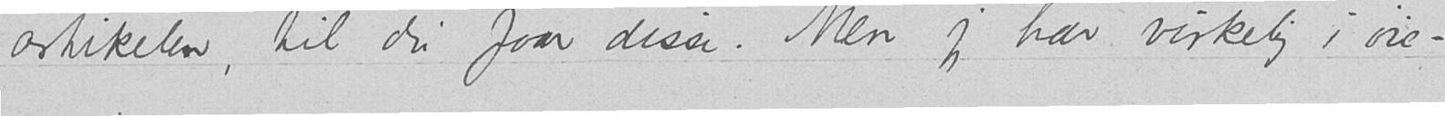artikelen, til du faar disse. Men jeg har virkelig i øie-
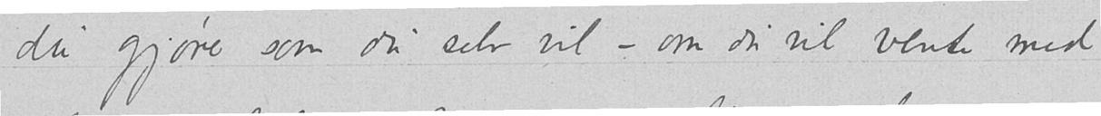du gjøre som du selv vil — om du vil vente med
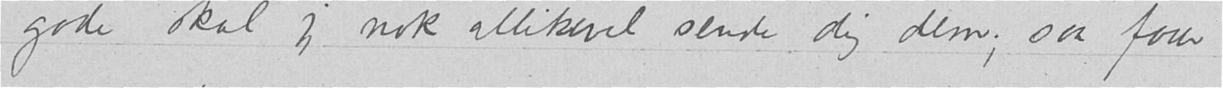gode skal jeg nok allikevel sende dig dem, saa faar
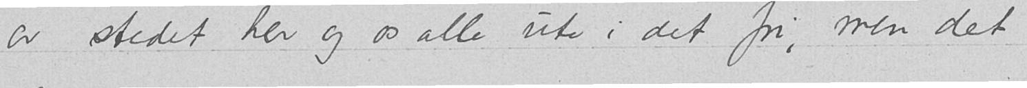av stedet her og os alle ute i det fri, men det
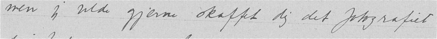men jeg vilde gjerne skaffet dig det fotografiet
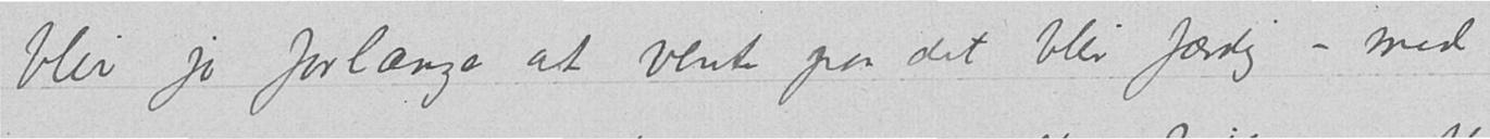blir jo forlænge at vente paa det blir færdig — med
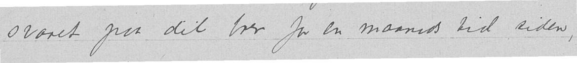svaret paa dit brev for en maaneds tid siden,
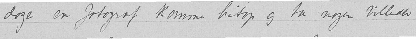dage en fotograf komme hitop og ta nogen billeder
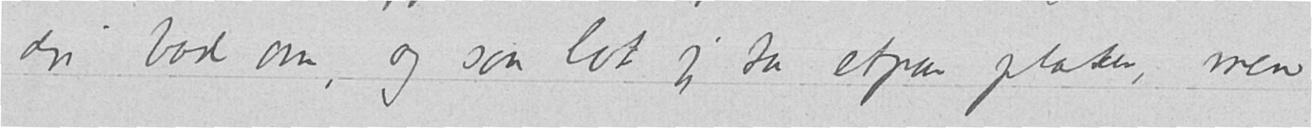du bad om, og saa lot jeg ta etpar plater, men
